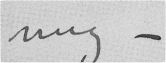mig –
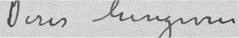Deres hengivne
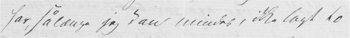har sålænge jeg kan mindes, ikke lagt to
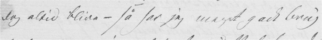dog altid blive – så har jeg meget godt Brug
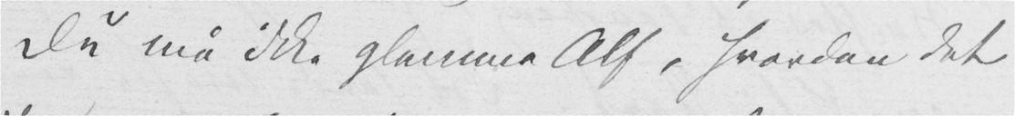Du må ikke glemme Alf, hvordan det
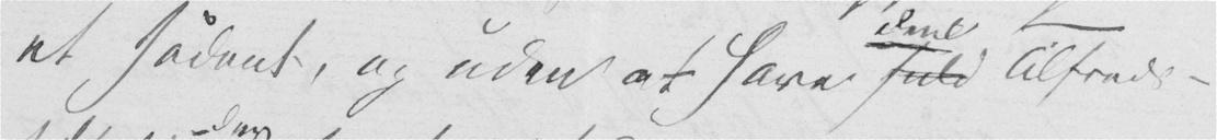et sådant, og uden at have fuld Tilfreds-
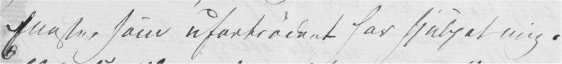Eneste, som ufortrødent har hjulpet mig.
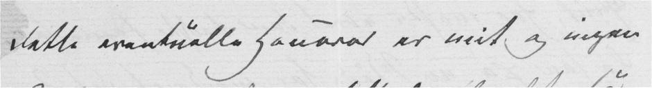Dette eventuelle Honorar er mit og ingen
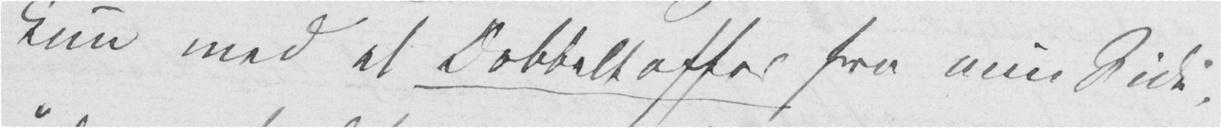kun med et Dobbeltoffer fra min Side,
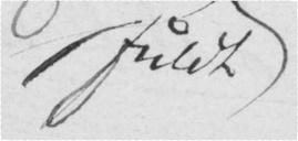fuldt
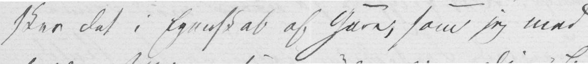sker det i Egenskab af Gave, som jeg med
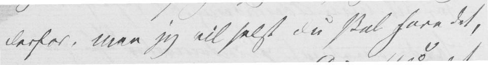derfor, men jeg vil helst Du skal have det,
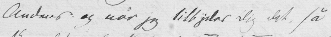Andens og når jeg tilbyder Dig det, så
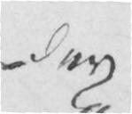der
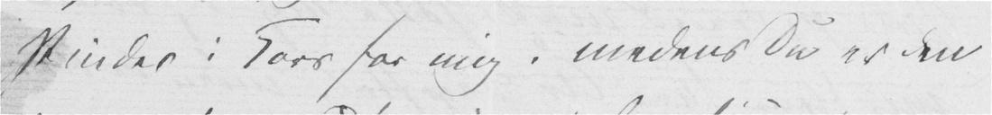Pinder i Kors for mig, medens Du er den
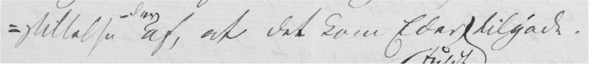stillelse af, at det kom Eder tilgode.
